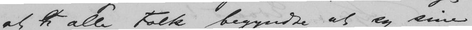at alle Folk begyndte at sy sine
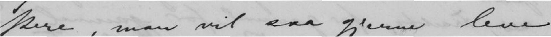stere, man vil saa gjerne leve
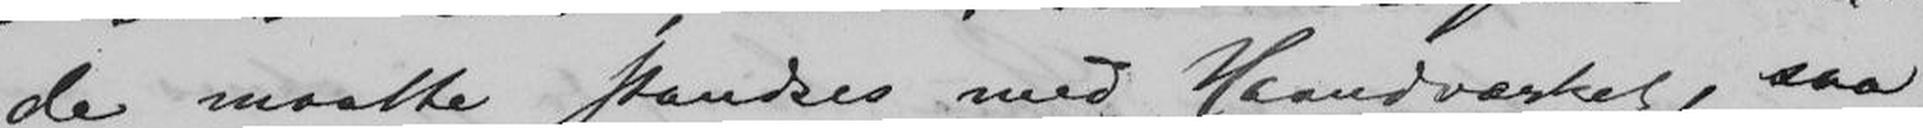de maatte standses med Haandværket, saa
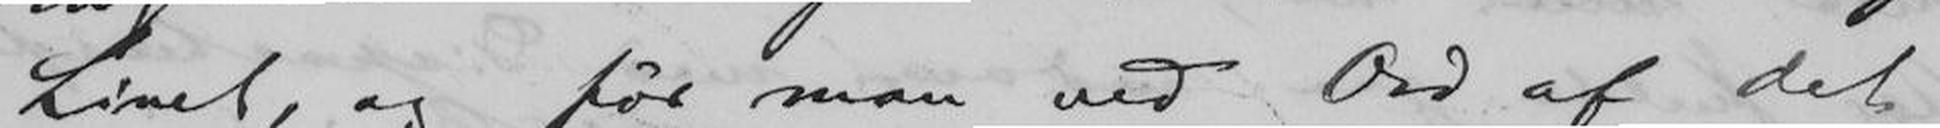Livet, og før man ved Ord af det,
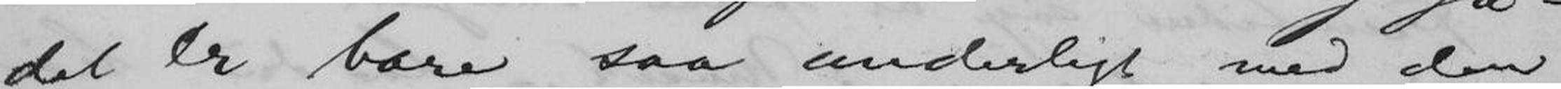det er bare saa underligt med den
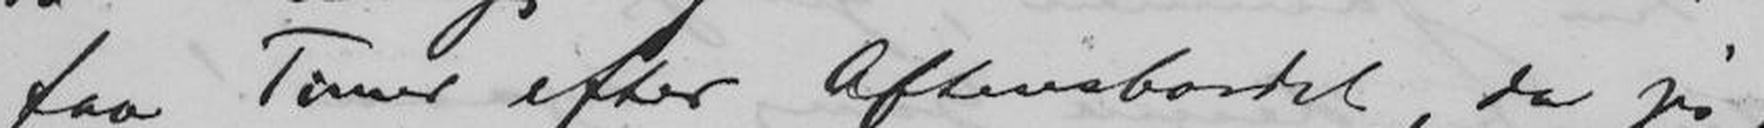faa Timer efter Aftensbordet, da jeg
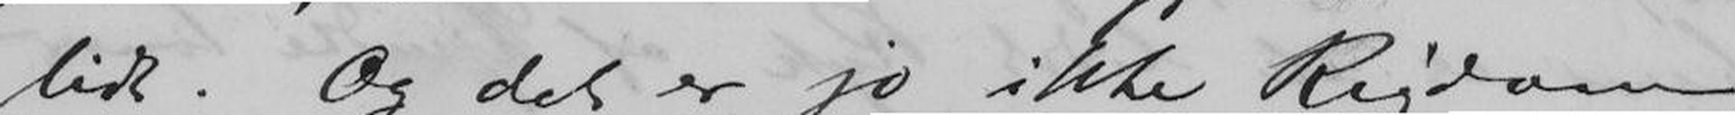lidt. Og det er jo ikke Rigdom
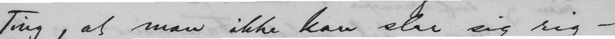Ting, at man ikke kan slaa sig rig-
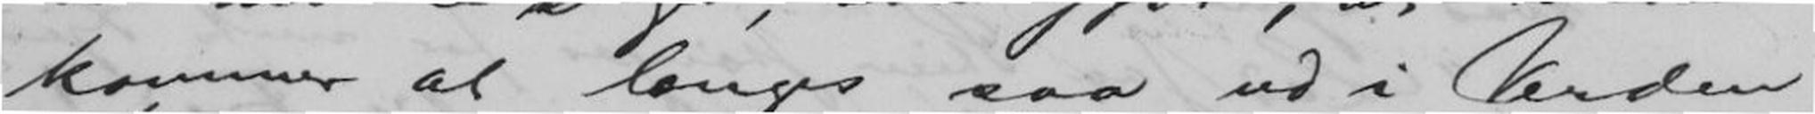kommer at længes saa ud i Verden
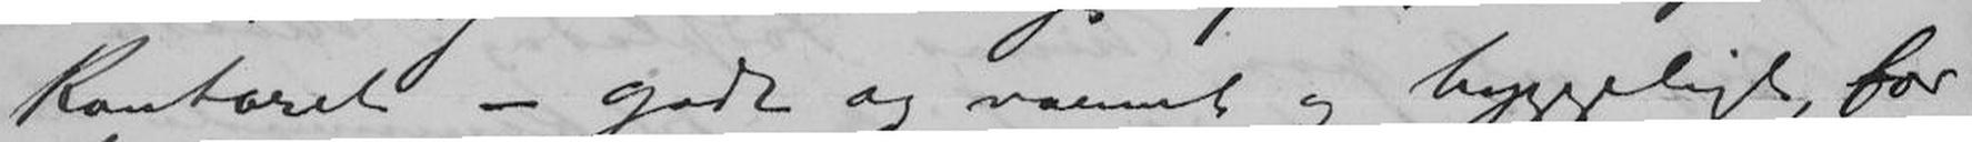Kontoret - godt og varmt og hyggeligt, for
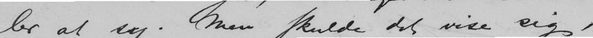ler at sy. Men skulde det vise sig,
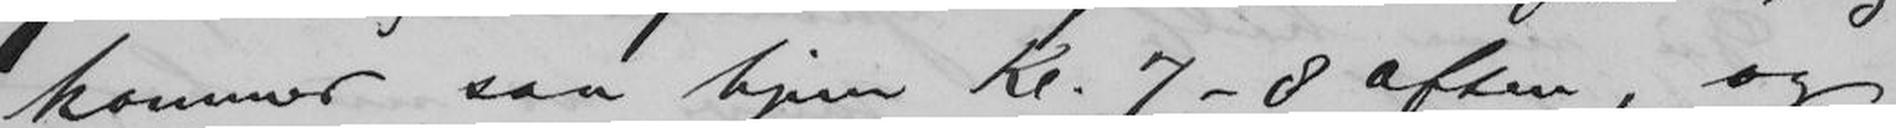kommer saa hjem Kl. 7 - 8 aften, og
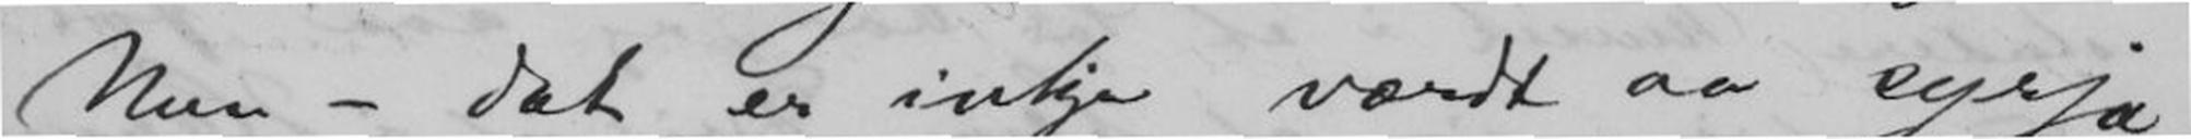Men - det er inkje værdt aa syrja -
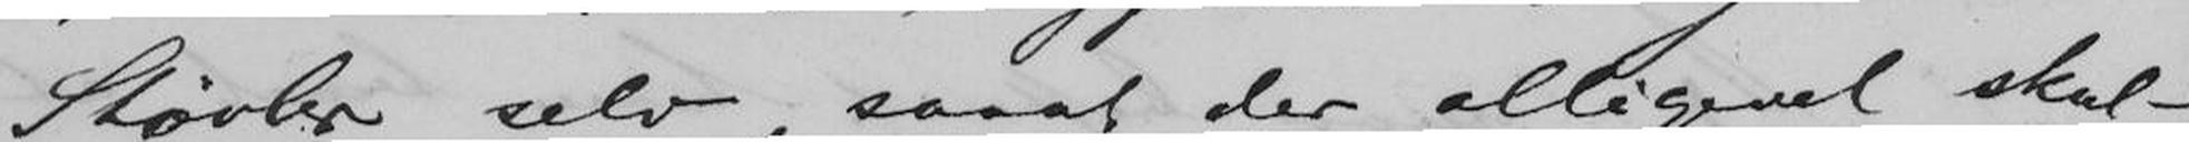Støvler selv, saaat der alligevel skul-
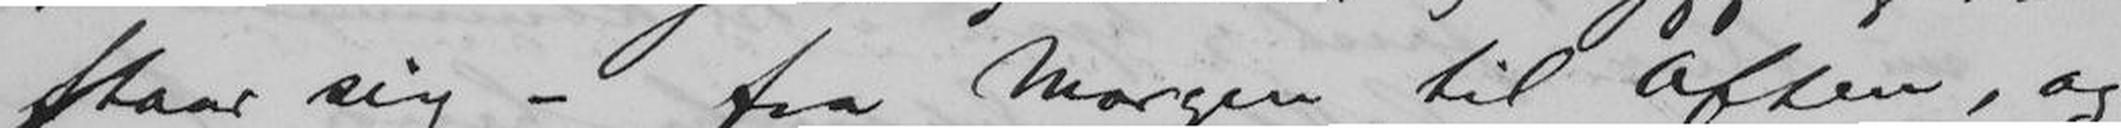staar sig - fra Morgen til Aften, og
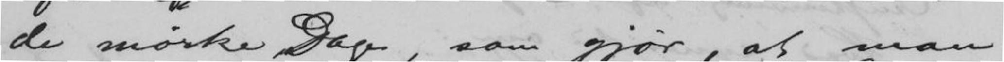de mørke Dage, som gjør, at man
